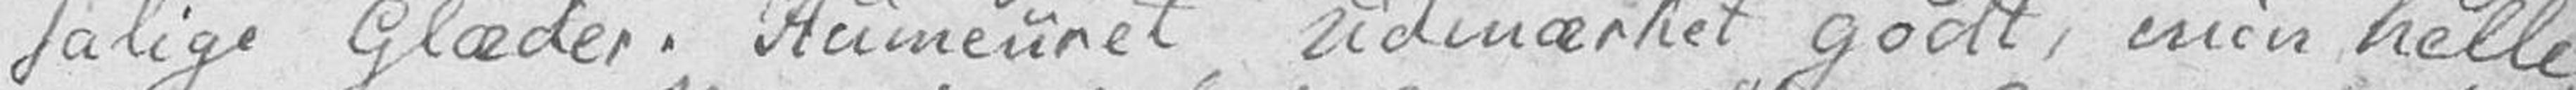salige Glæder. Humeuret Udmærket godt, min helle
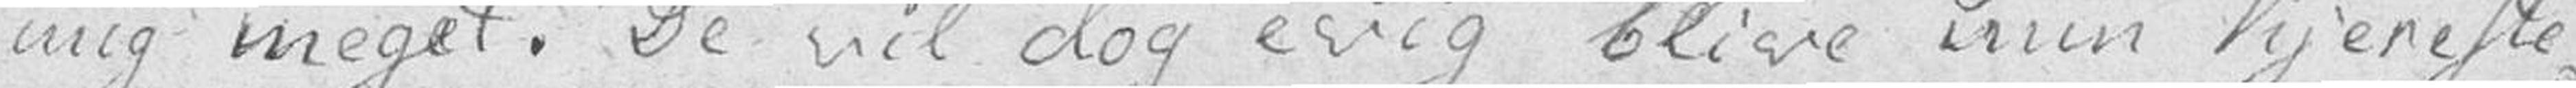mig meget. De vil dog evig blive min Kjereste
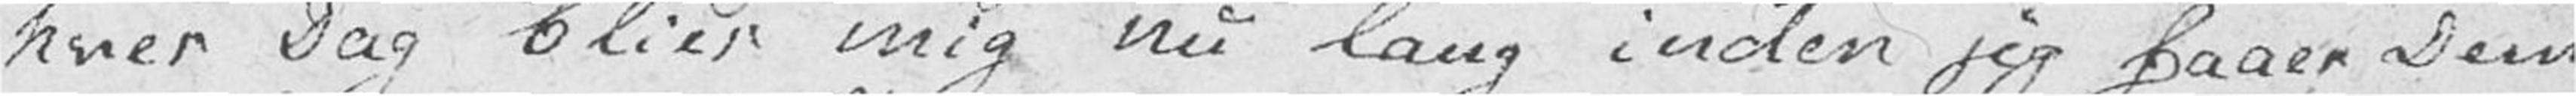hver Dag blier mig nu lang inden jeg faaer Dem
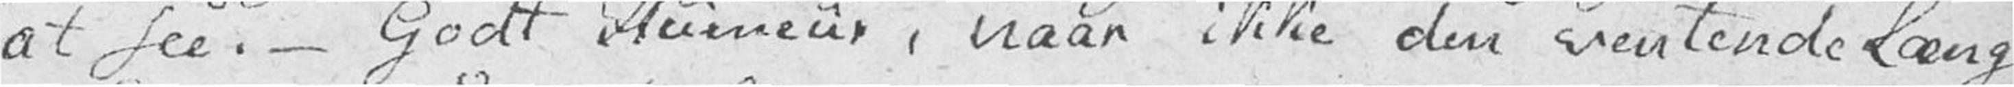at see. – Godt Humeur, naar ikke den ventende Læng
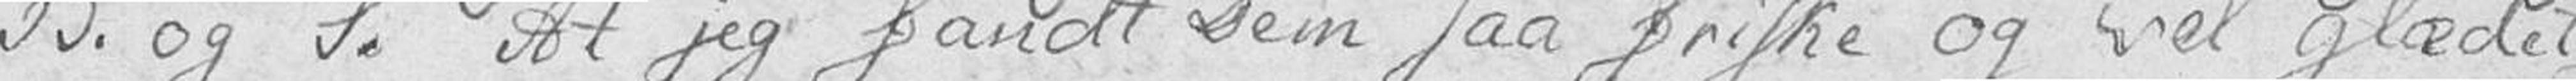B. og S. At jeg fandt Dem saa friske og vel glædet
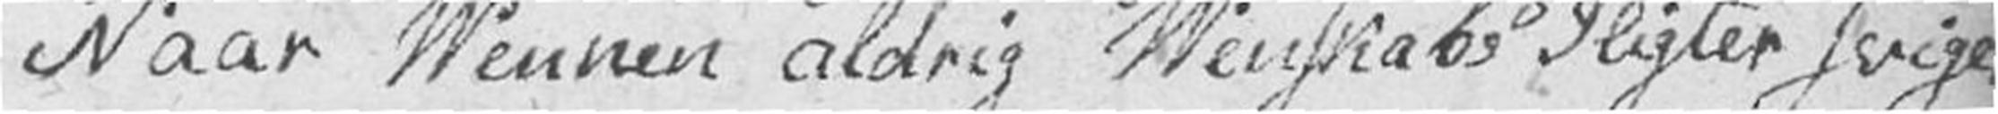Naar Vennen aldrig Venskabs Pligter svige.
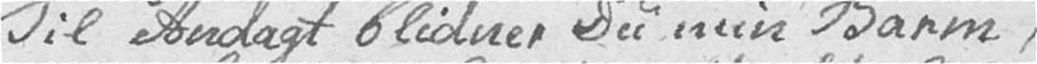Til Andagt blidner Du min Barm,
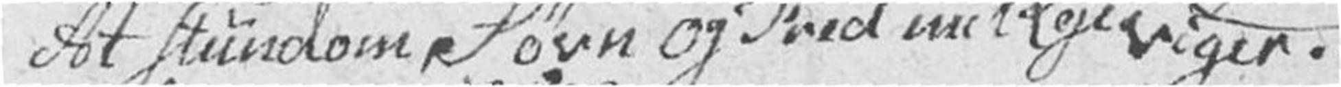At stundom Søvn og Fred mit Leje viger.
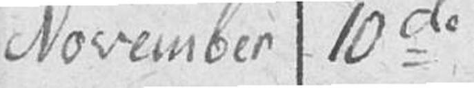November 10de
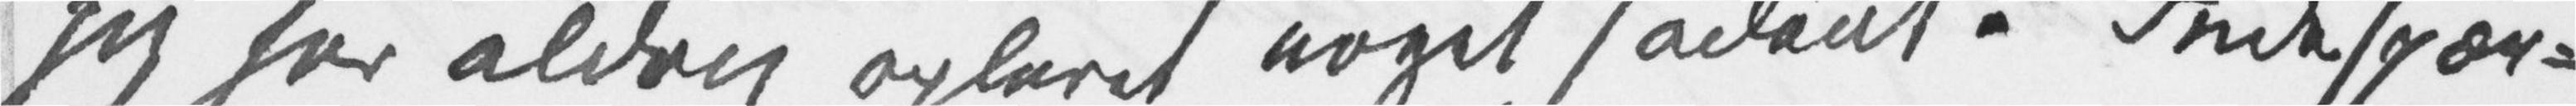jeg har aldrig oplevet noget sådant. Indespær¬
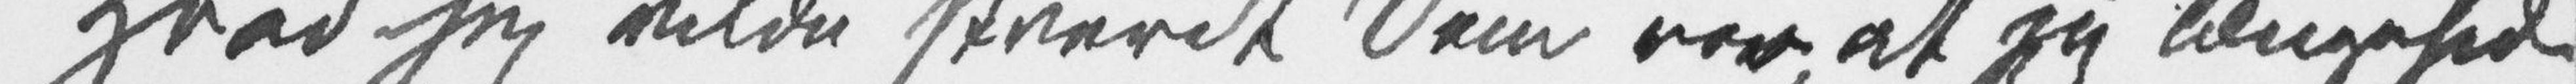Hvad jeg vilde skrevet Dem var, at jeg længesid[en]
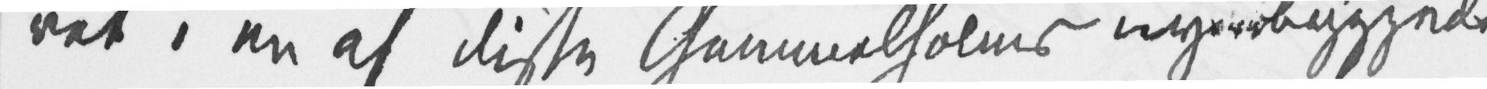ret i en af disse Gammelholms ny–byggede
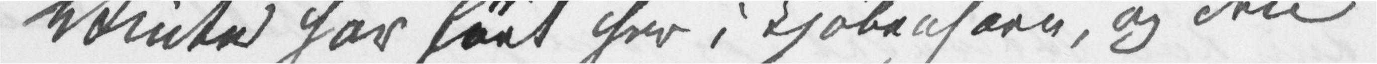Vinter har ført her i Kjøbenhavn, og den
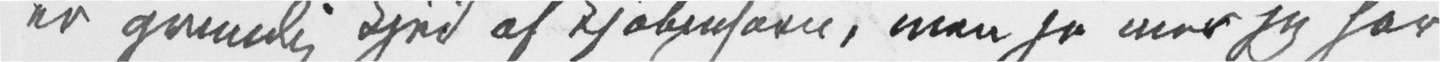er grundig kjed af Kjøbenhavn, men jo mer jeg har
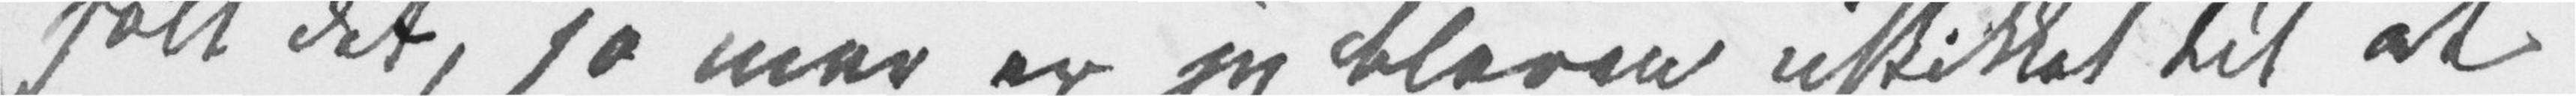følt det, jo mer er jeg bleven uskikket til at
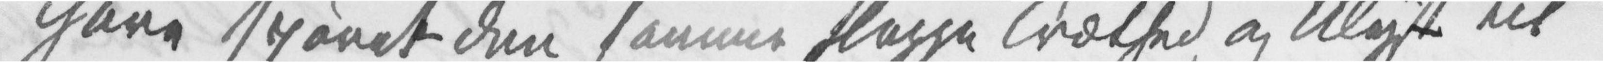have sporet den samme slappe Træthed og Ulyst til
